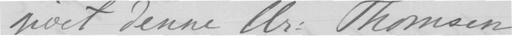givet denne Hr. Thomsen
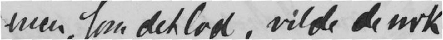men, som det lod, vilde de nok
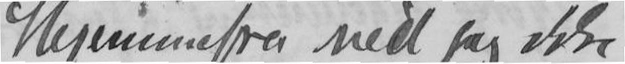Hjemmefra ved jeg ikke
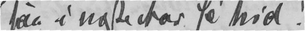I saa i nogle Aar Se hid!
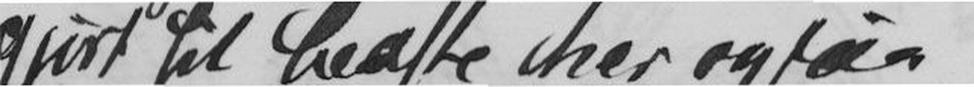gjort sit bedste her ogsaa.
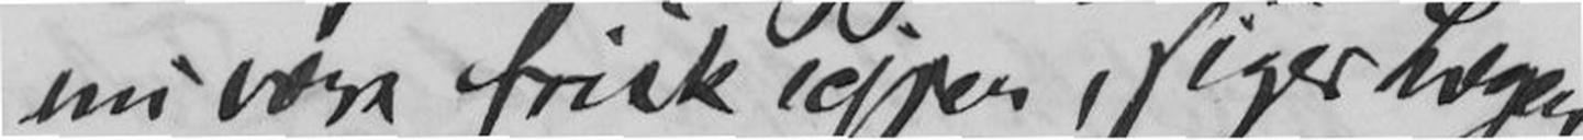nu være frisk igjen, siger Lægen,
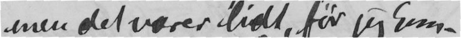men det varer lidt, før jeg kom-
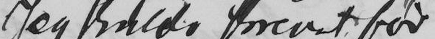Jeg skulde skrevet før,
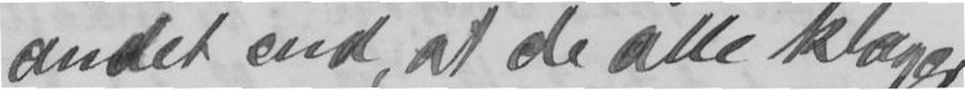andet end, at de alle klager
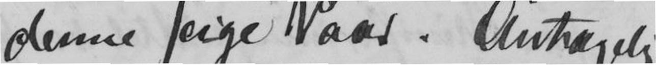denne seige Vaar. Antagelig
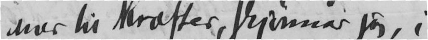mer til Kræfter, skjønner jeg, i
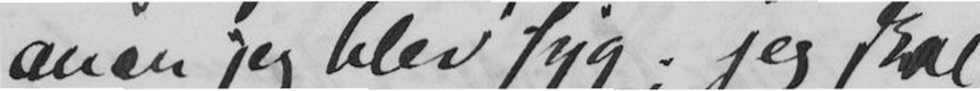men jeg blev syg; jeg skal
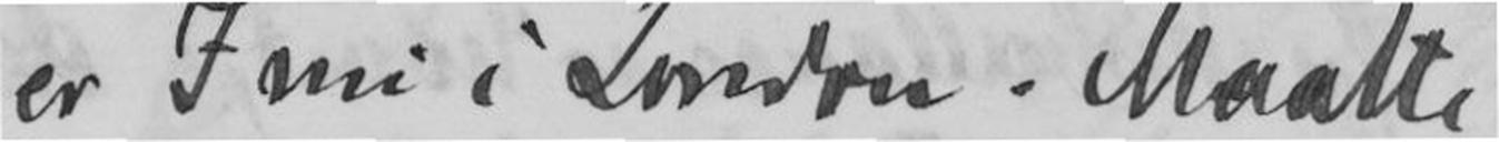er I nu i London. Maatte
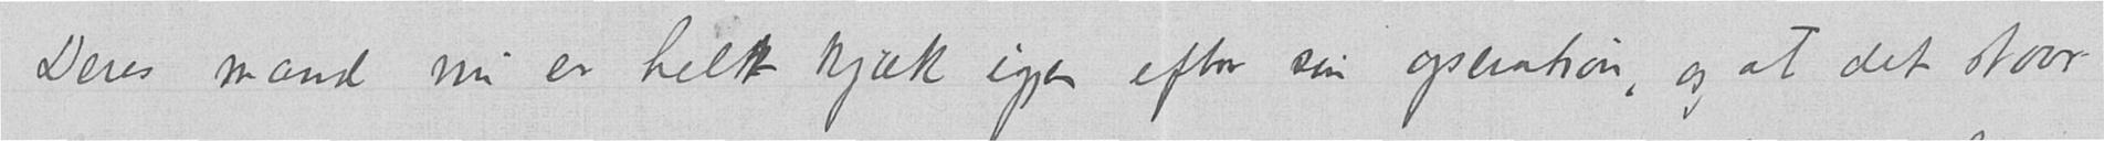Deres mand nu er helt kjæk igjen efter sin operation, og at det staar
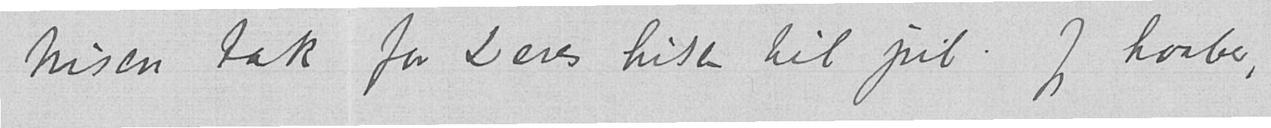tusen tak for Deres hilsen til jul. Jeg haaber,
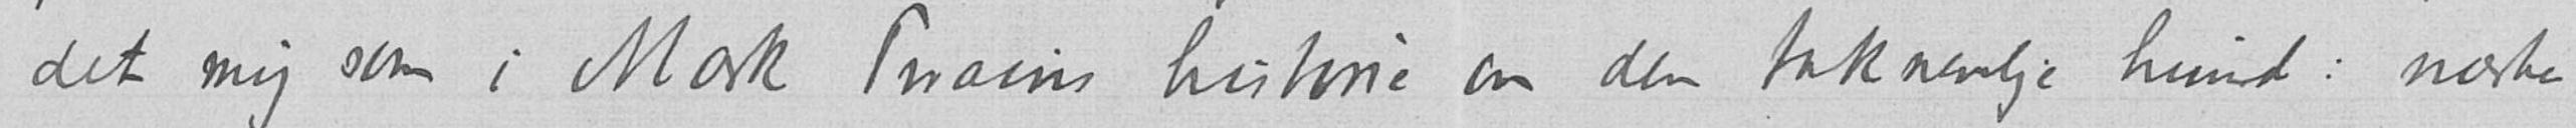det mig som i Mark Twains historie om den taknemlige hund: næste
